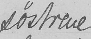søstrene
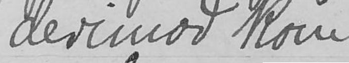derimod kom
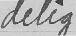delig
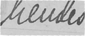hendes
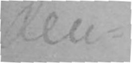den-
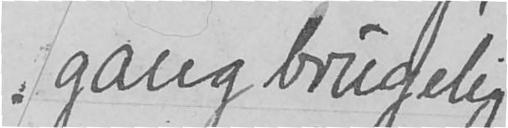gang brugelig
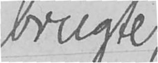brugte,
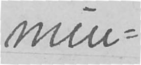min-
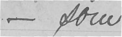- som
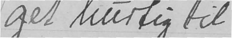get hurtig til
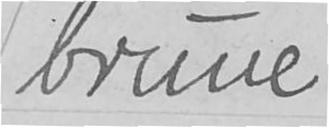brune
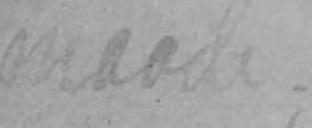maade;
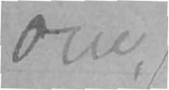om,
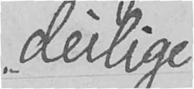deilige
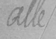alle
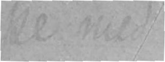pe med
